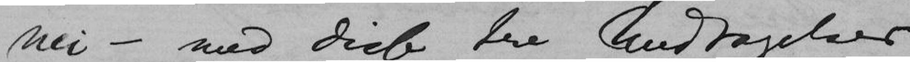nei - med disse tre Undtagelser
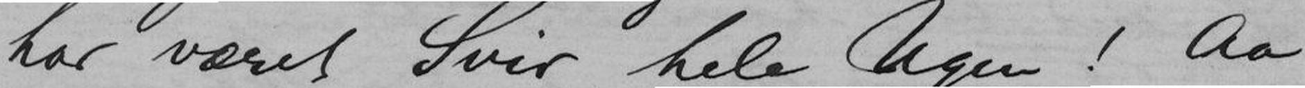har været Svir hele Ugen! Aa
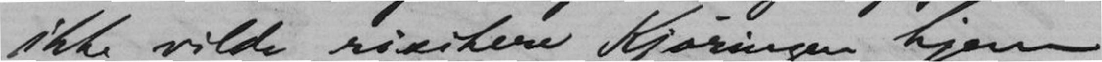ikke vilde risikere Kjøringen hjem
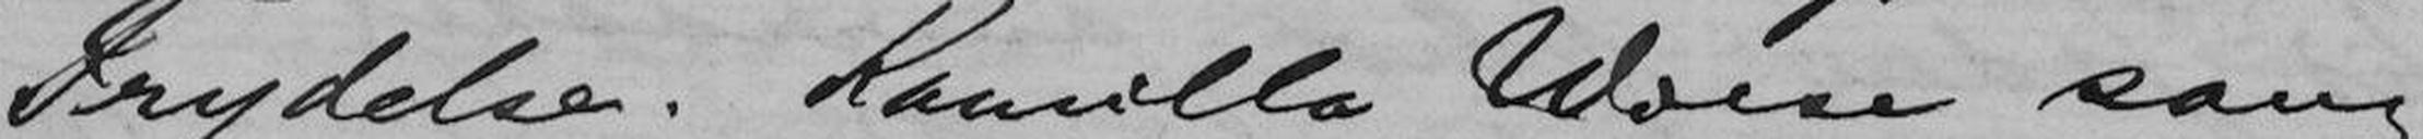Frydelse. Kamilla Wiese sang
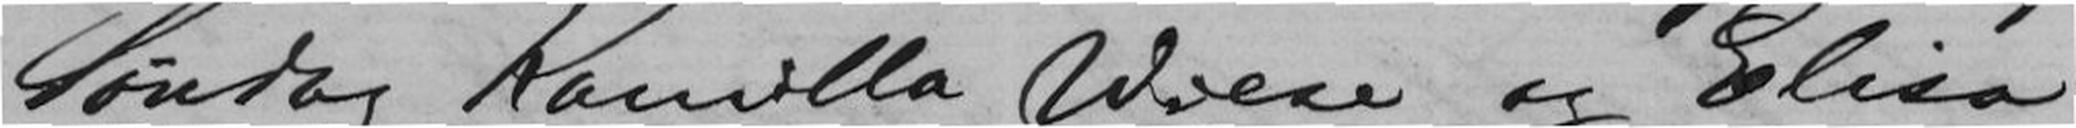Søndag Kamilla Wiese og Elisa
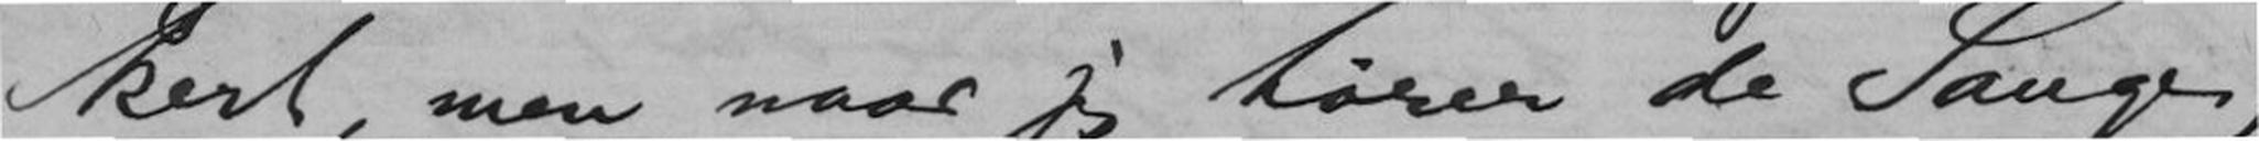kert, men naar jeg hører de Sange,
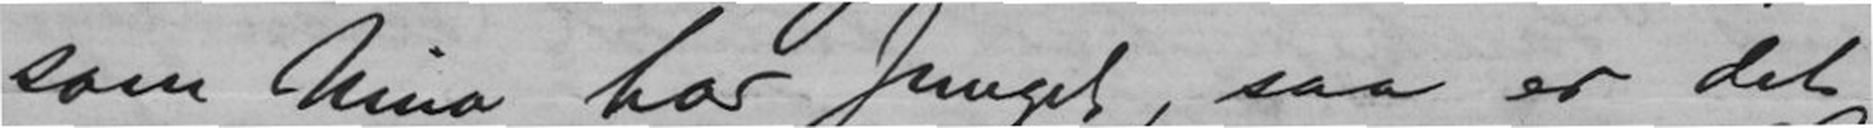som Nina har sunget, saa er det
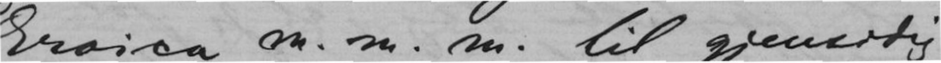Eroica m.m.m. til gjensidig
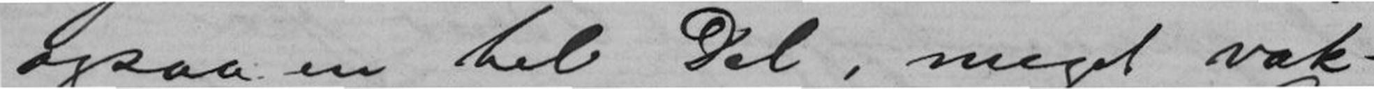ogsaa en hel Del, meget vak-
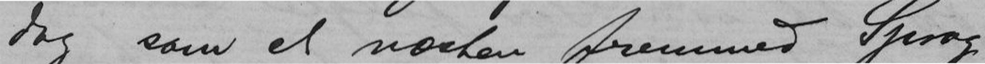dog som et næsten fremmed Sprog
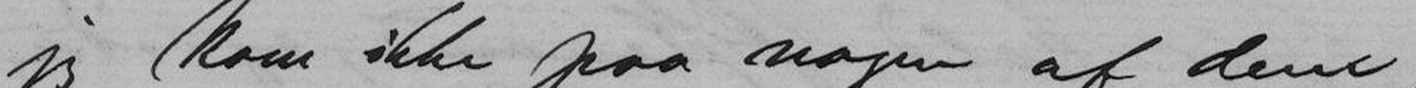jeg kom ikke paa nogen af dem,
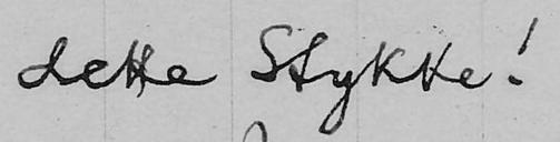dette Stykke!
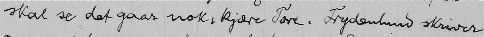skal se det gaar nok, kjære Tore. Frydenlund skriver
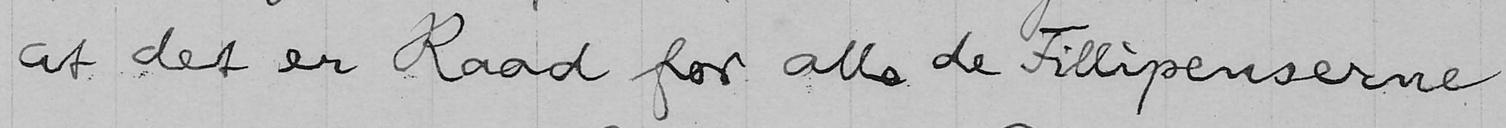at det er Raad for alle de Fillipenserne
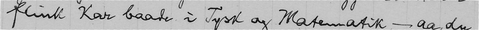flink Kar baade i Tysk og Matematik - aa, du
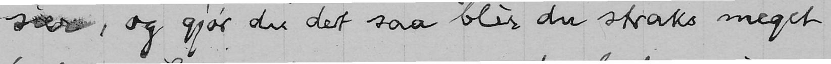sier, og gjør du det saa blir du straks meget
